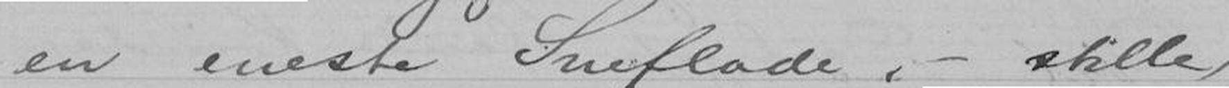en eneste Sneflade, - stille,
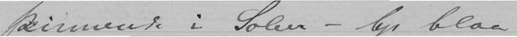skinnende i Solen - lys blaa
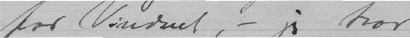for Vinduet, - jeg tror
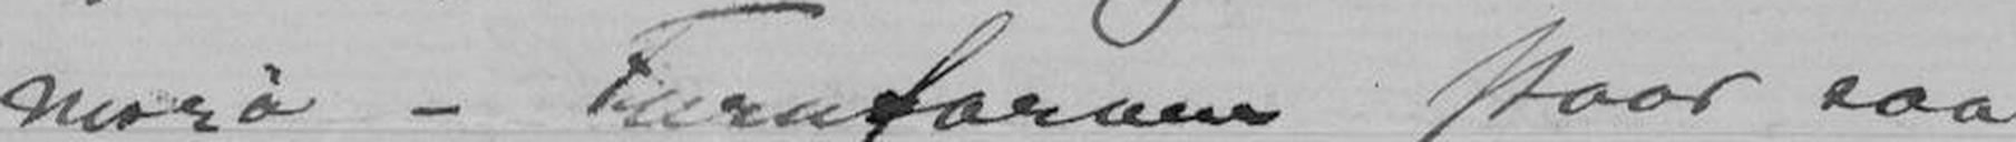morø - Furufarven staar saa
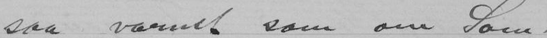saa varmt som om Som-
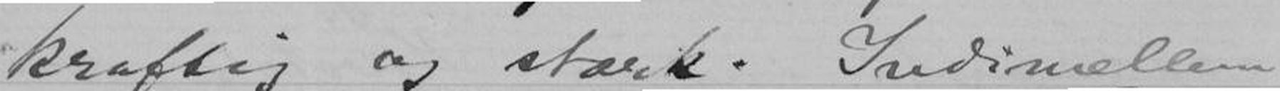kraftig og stærk. Indimellem
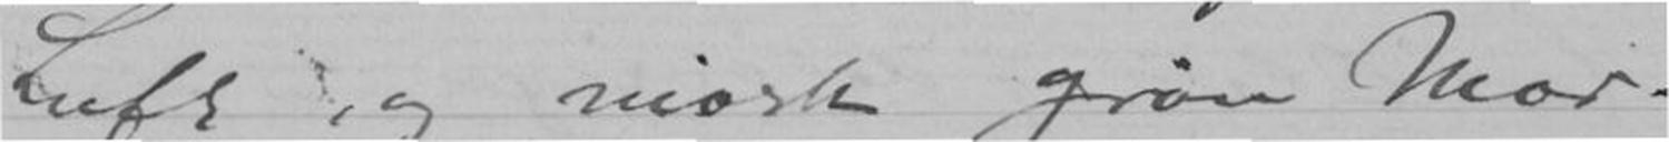Luft, og mørk grøn Mar-
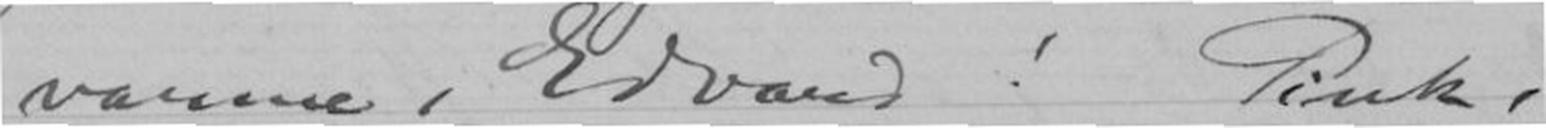varme, Edvard! Pink,
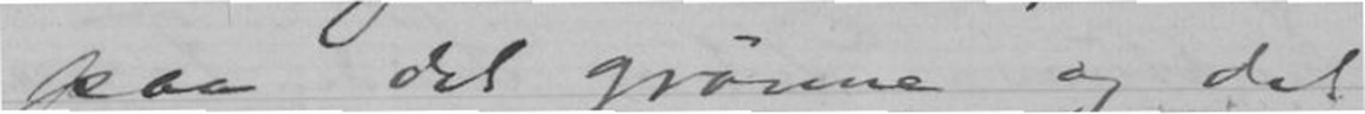paa det grønne og det
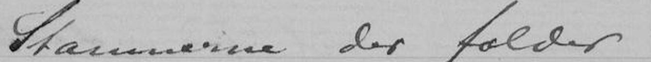Stammerne der falder Solen
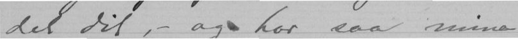det Dit, - og har saa mine
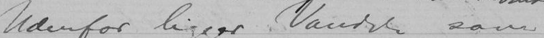Udenfor ligger Vandet som
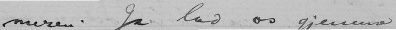meren. Ja lad os gjemme
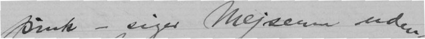pink - siger Mejserne uden-
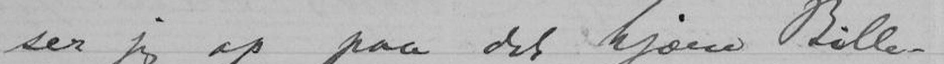ser jeg op paa det kjære Bille-
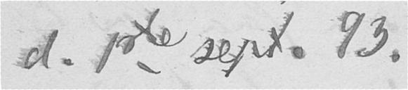d. 1ste sept. 93.
